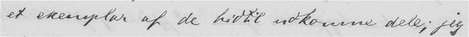et exemplar af de hidtil udkomne dele; jeg
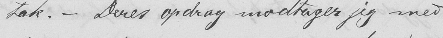tak. - Deres opdrag modtager jeg med
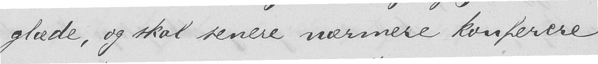glæde, og skal senere nærmere konferere
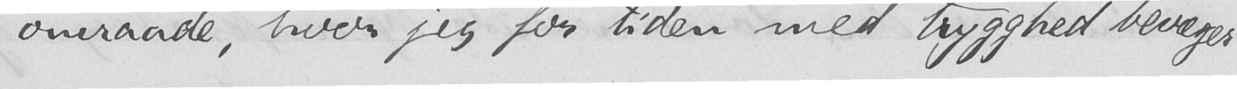omraade, hvor jeg for tiden med trygghed bevæger
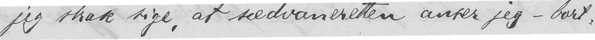jeg strax sige, at sædvaneretten anser jeg - bort-
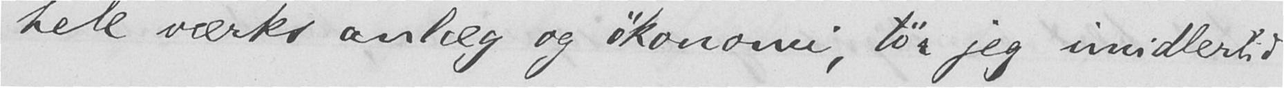hele værks anlæg og økonomi, tør jeg imidlertid
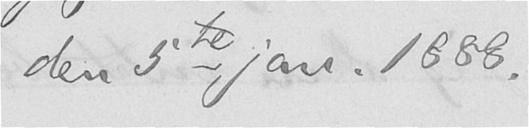den 5te jan. 1888.
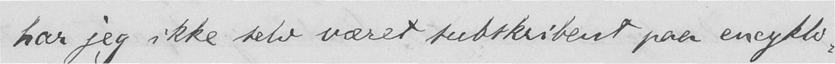har jeg ikke selv været subskribent paa encyklo-
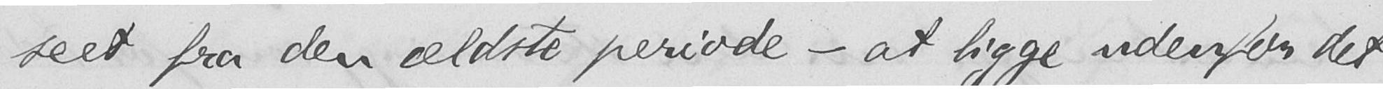seet fra den ældste periode - at ligge udenfor det
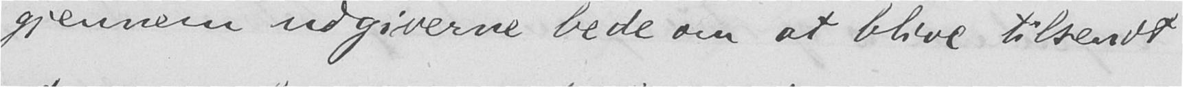gjennem udgiverne bede om at blive tilsendt
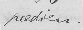pædien.
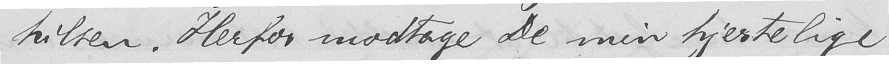hilsen. Herfor modtage De min hjertelige
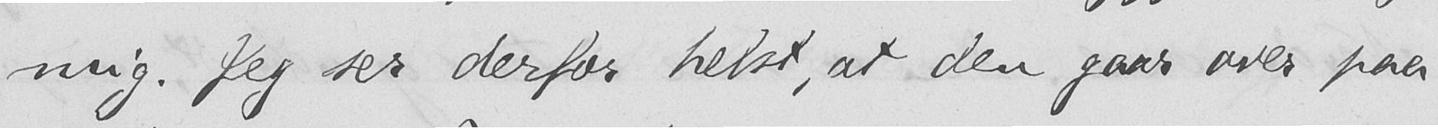mig. Jeg ser derfor helst, at den gaar over paa
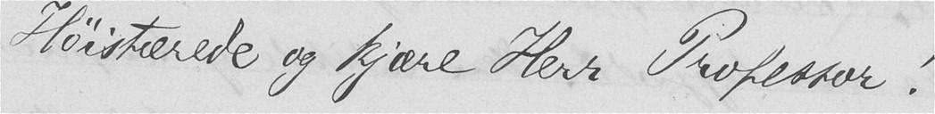Høistærede og kjære Herr Professor!
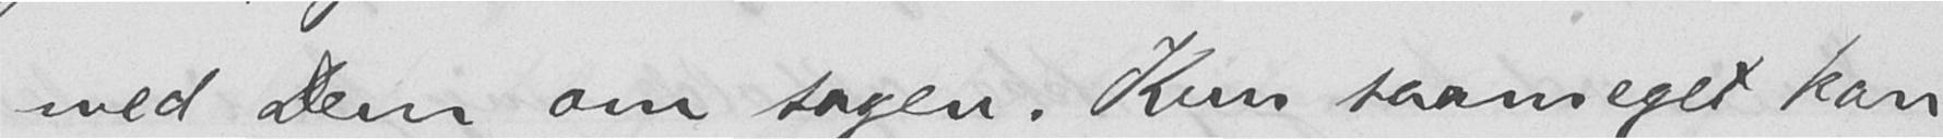med Dem om sagen. Kun saameget kan
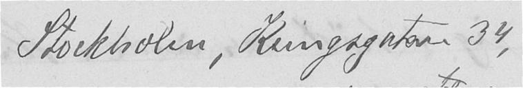Stockholm, Kungsgatan 34,
